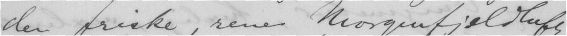den friske, rene Morgenfjeldluft
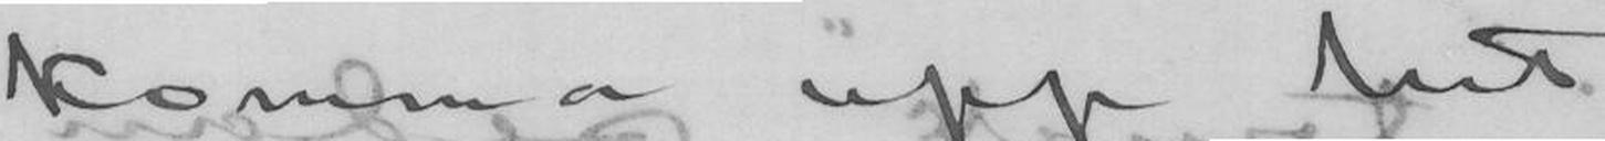komma upp hid
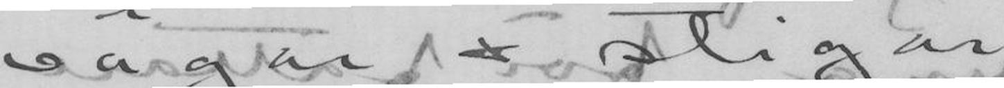vägar & stigar
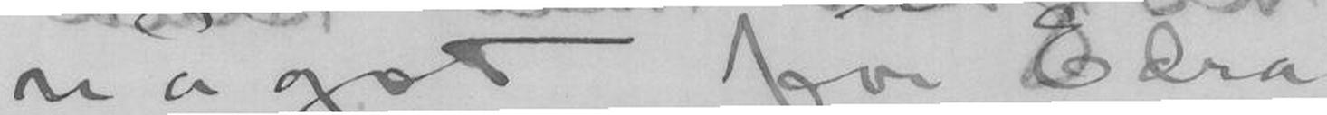något for Edra
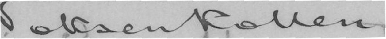Voksenkollen
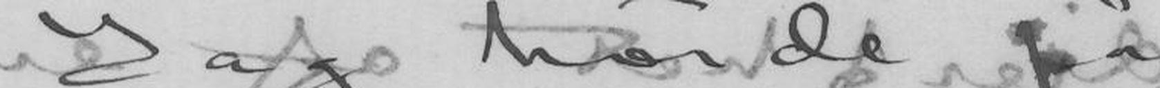Jag hørde på
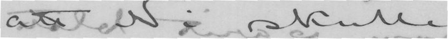att Ni skulle
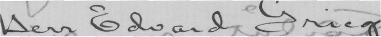Herr Edvard Grieg,
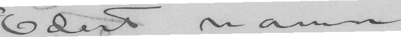Edert namn
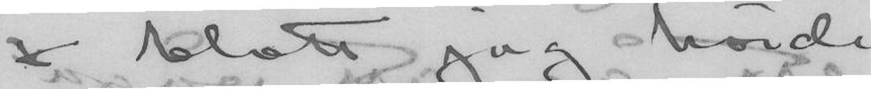& blott jag hörde
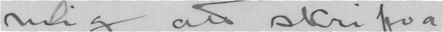mig att skri fva
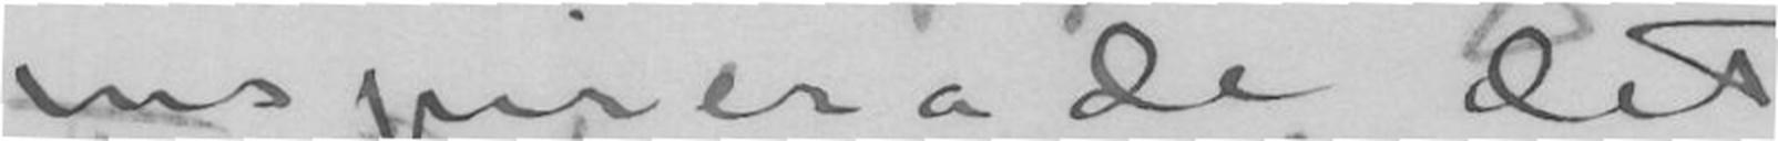inspirerade det
